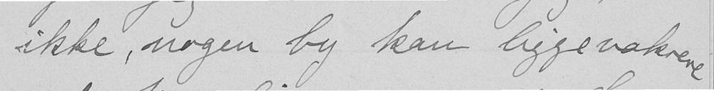ikke, nogen by kan ligge vakrere
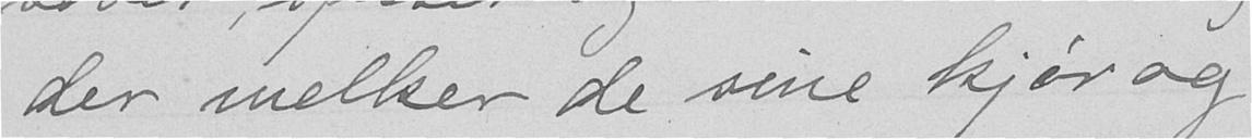der melker de sine kjør og
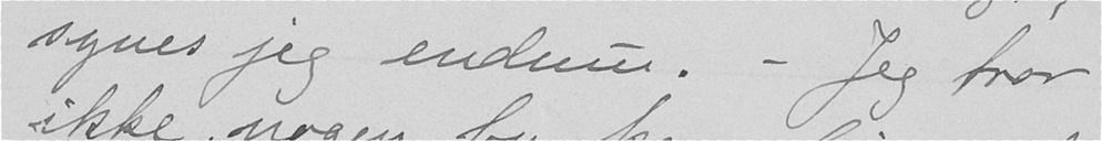synes jeg endnu. - Jeg tror
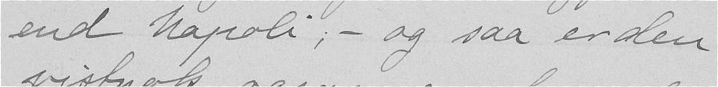end Napoli, - og saa er den
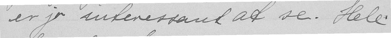er jo interessant at se. Hele
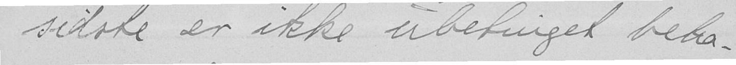sidste er ikke ubetinget beha-
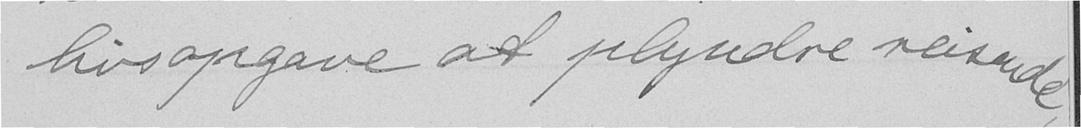livsopgave at plyndre reisende
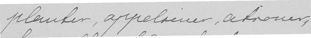planter, appelsiner, sitroner,
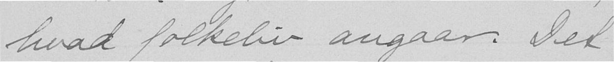hvad folkeliv angaar. Det
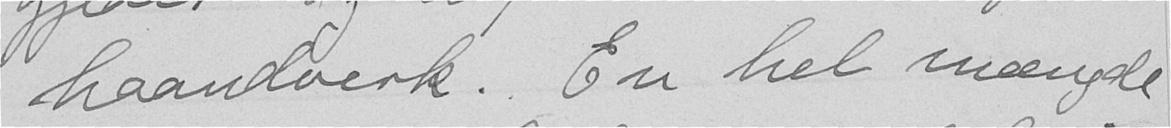haandverk. En hel mængde
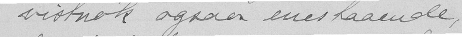vistnok ogsaa enestaaende
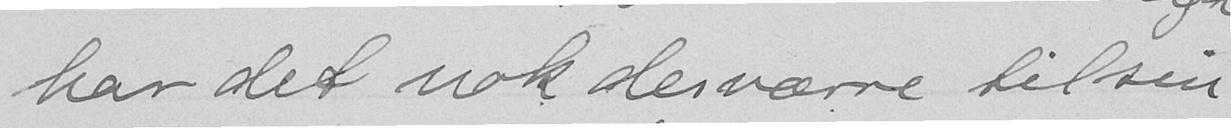har det nok desværre til sin
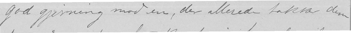god gjerning mod en, der allerede takker dem
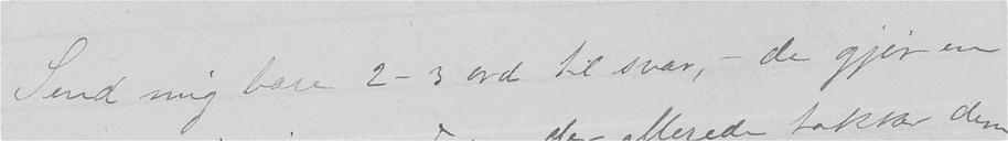Send mig bare 2-3 ord til svar, - de gjør en
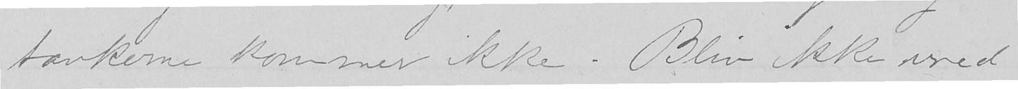tankerne kommer ikke. Bliv ikke vred
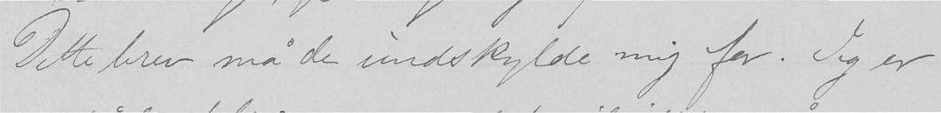Dette brev må de undskylde mig for. Jeg er
